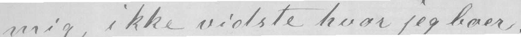mig, ikke vidste hvor jeg boer.
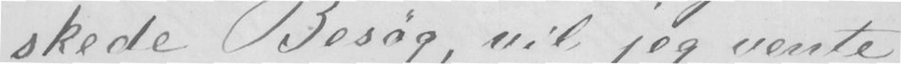skede Besøg, vil jeg vente,
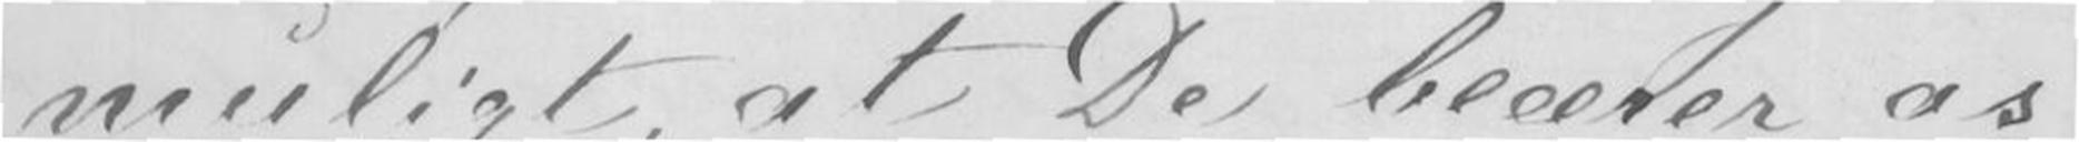muligt, at De beærer as
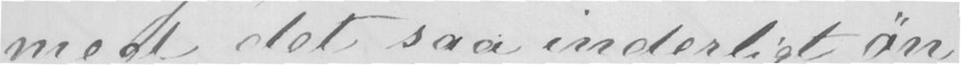med det saa inderligt øn-
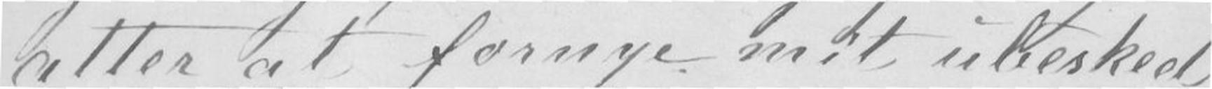atter at fornye mit ubesked-
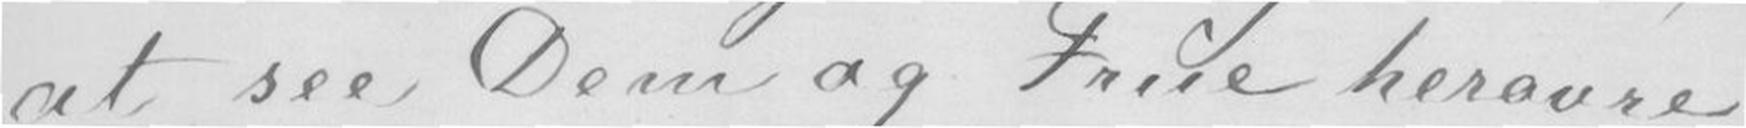at see Dem og Frue herovre,
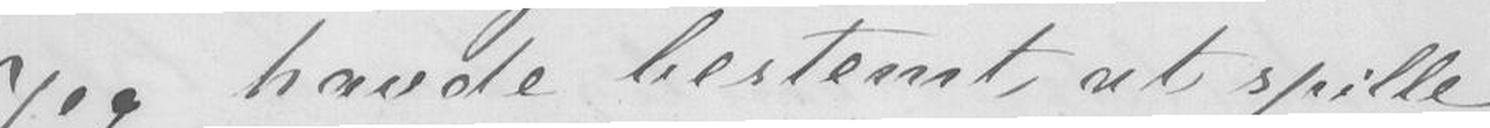Jeg havde bestemte at spille
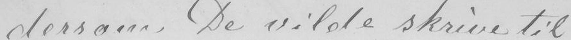dersom De vilde skrive til
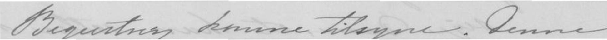Begeistring komme tilsyne. Denne
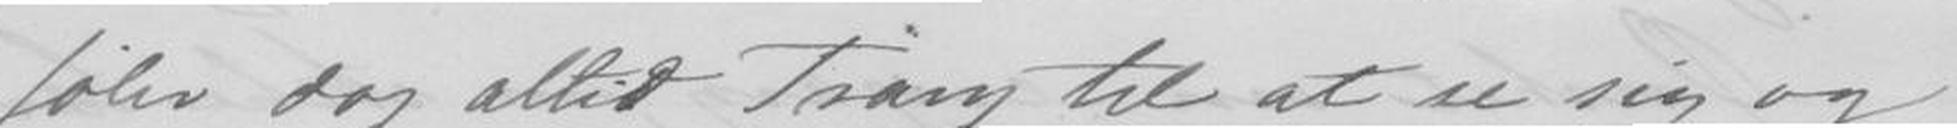føler dog altid Trang til at se sig og
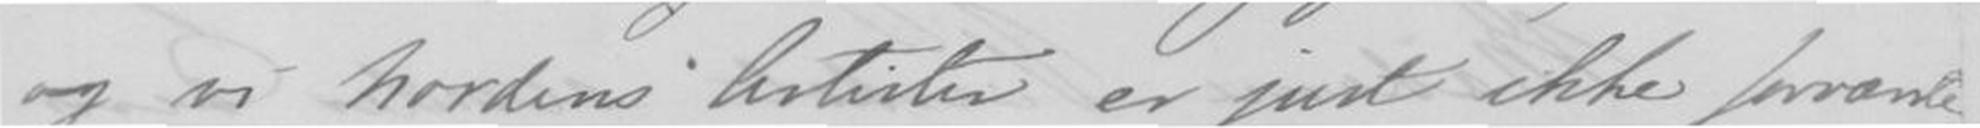og vi Nordens Artister er just ikke forvænte
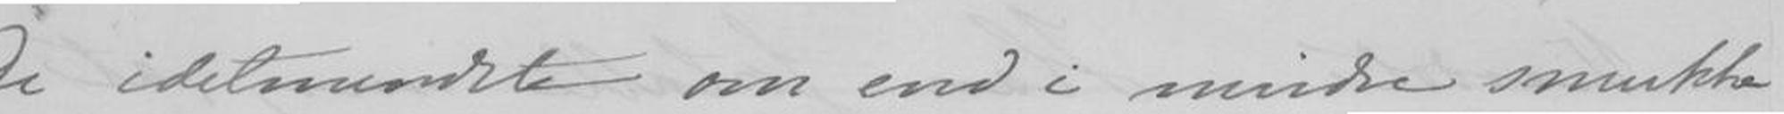De idetmindste om end i mindre smukke
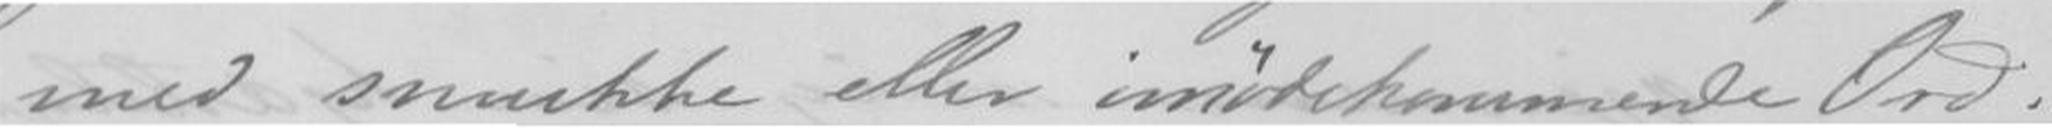med smukke eller imødekommende Ord.
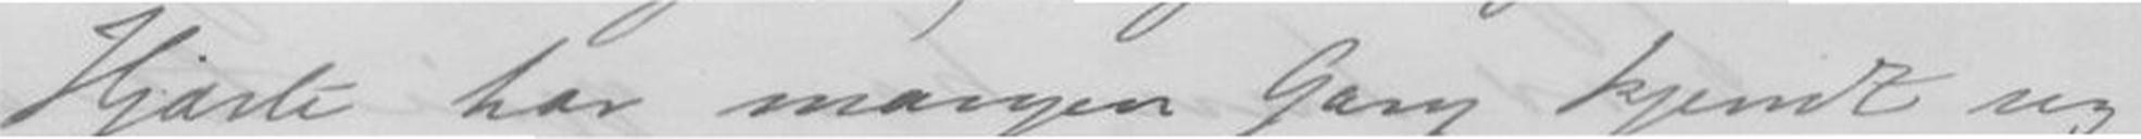Hjærte har mangen gang kjendt sig
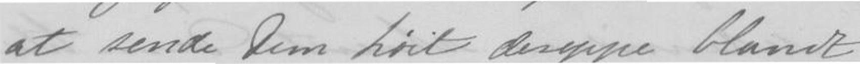at sende Dem høit deroppe blandt
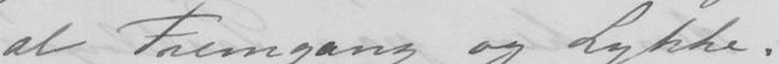al Fremgang og Lykke.
